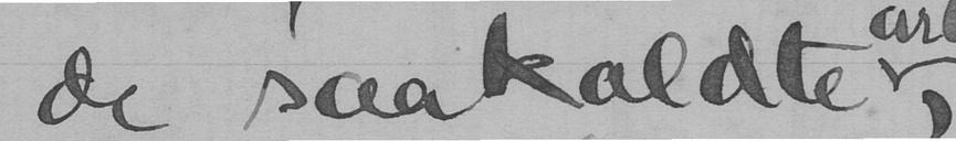de saakaldte
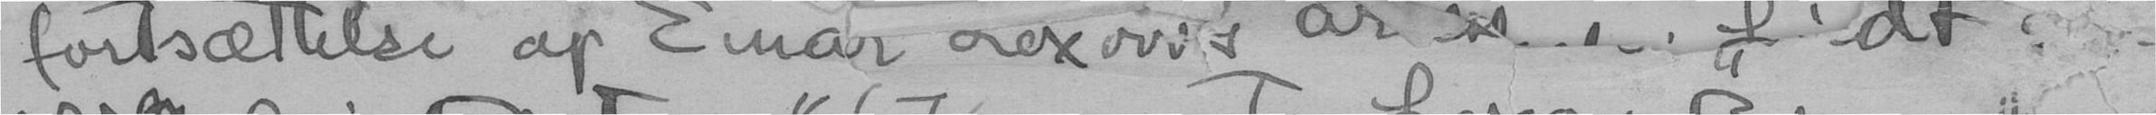fortsættelse af Einar Lexow's artikel: "Lidt om
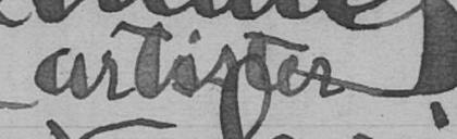artister
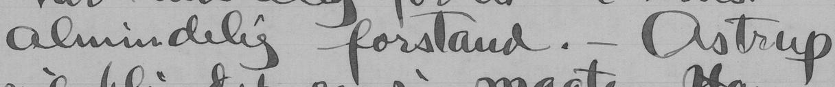almindelig forstand. - Astrup
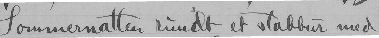Sommernatten rundt et stabbur med
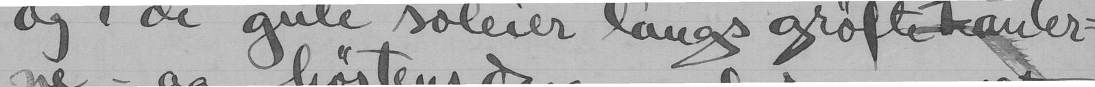og i de gule soleier langs grøftekanter-
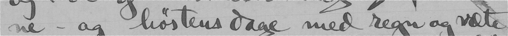ne - og høstens dage med regn og væte
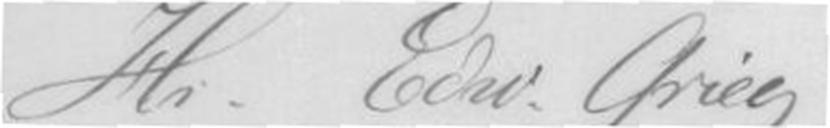Hr. Edw. Grieg
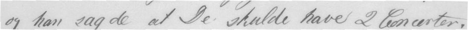og han sagde at De skulde have 2 Concerter.
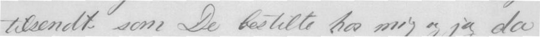tilsendt som De bestilte hos mig og jeg da
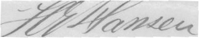HE.Hansen
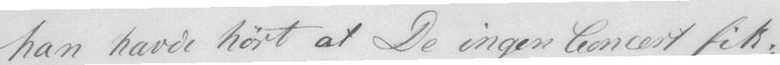han havde hørt at De ingen Concert fik;
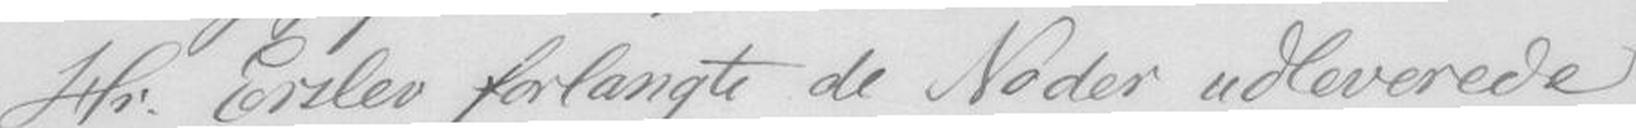Hr. Erslev forlangte de Noder udleverede
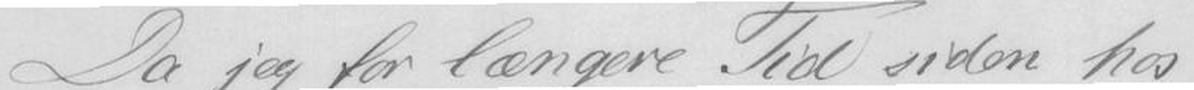Da jeg for længere Tid siden hos
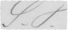S.T
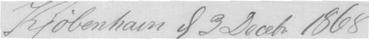Kjøbenhavn d 3 Decebr. 1868
